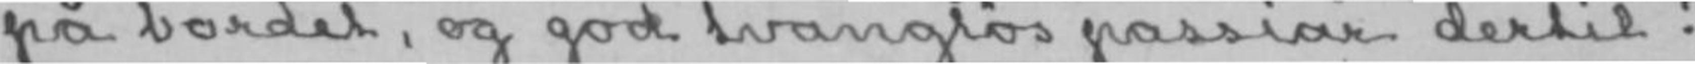på bordet, og god tvangløs passiar dertil!
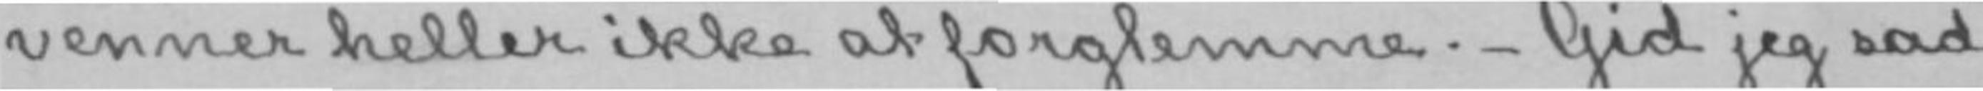venner heller ikke at forglemme.- Gid jeg sad
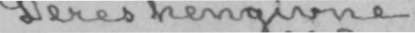Deres hengivne
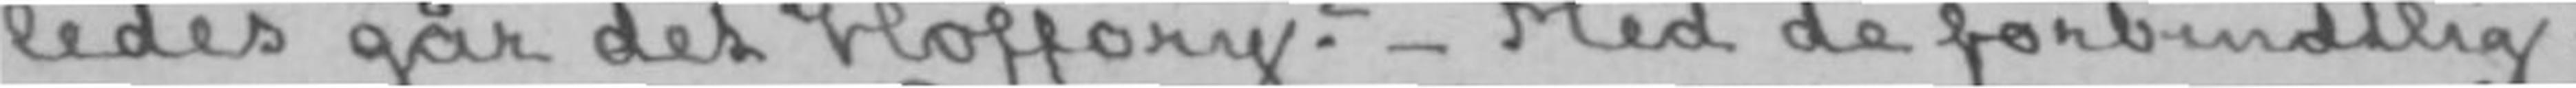ledes går det Hoffory?- Med de forbindtlig-
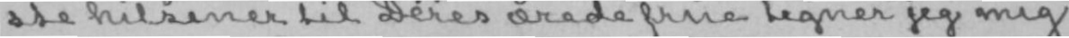ste hilsener til Deres ærede frue tegner jeg mig
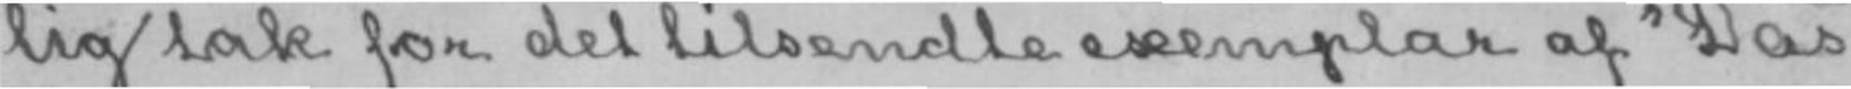lig tak for det tilsendte exemplar af «Das
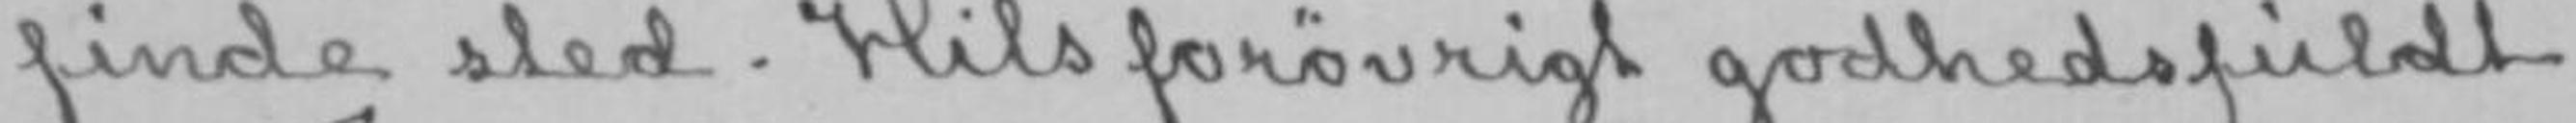finde sted. Hils forøvrigt godhedsfuldt
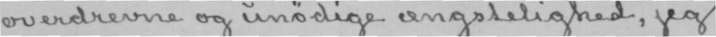overdrevne og unødige ængstelighed, jeg
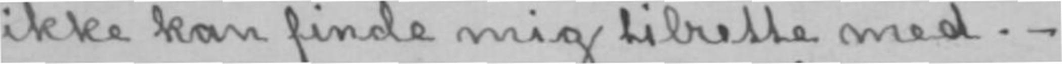ikke kan finde mig tilrette med.-
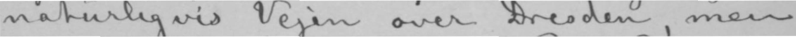naturligvis Vejen over Dresden, men
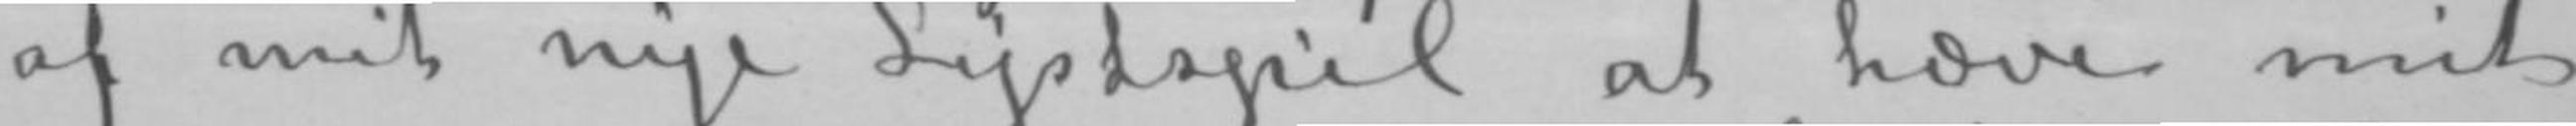af mit nye Lystspil at hæve mit
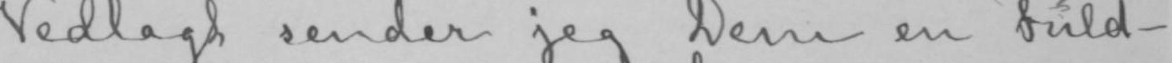Vedlagt sender jeg Dem en Fuld-
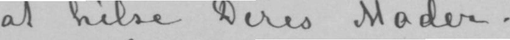at hilse Deres Moder.
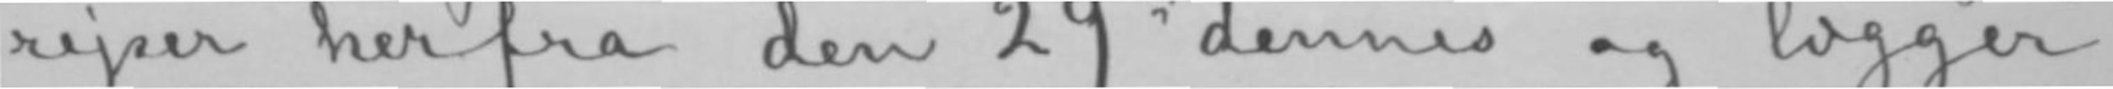rejser herfra den 29de dennes og lægger
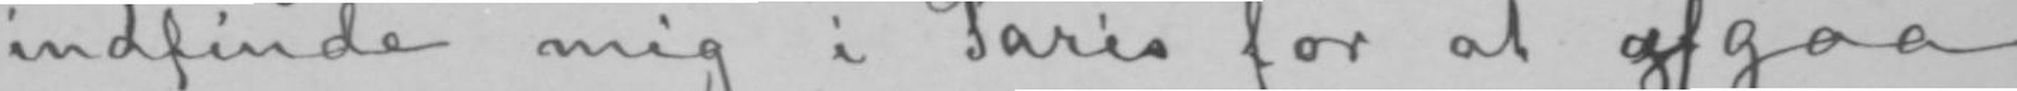indfinde mig i Paris for at afgaag
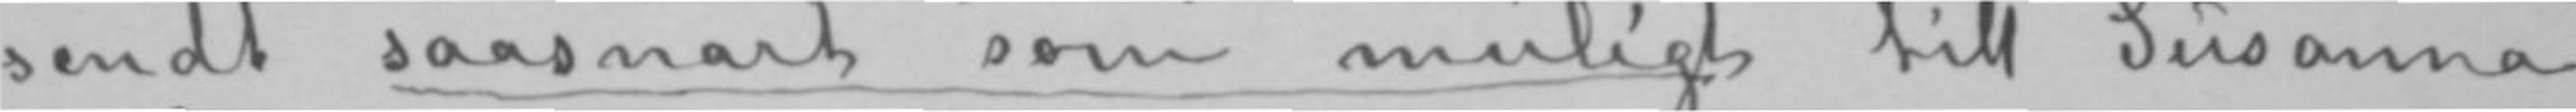sendt saasnart som muligt till Susanna
